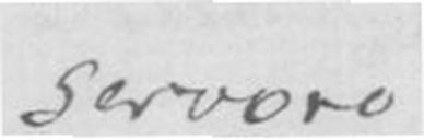servere
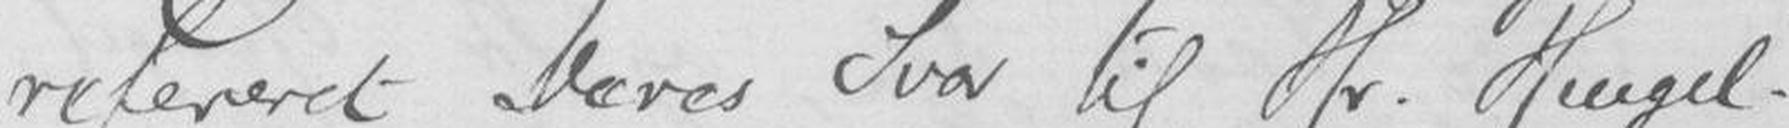refereret Deres Svar til Hr. Heugel
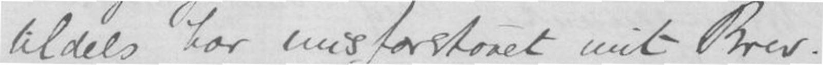tildels har misforstaaet mit Brev.
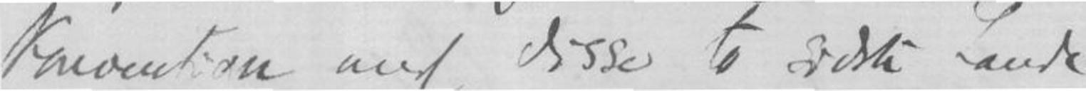med disse to Lande
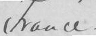France
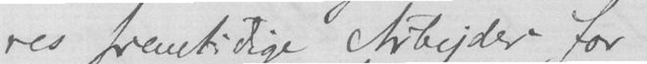res fremtidige Arbeijder for
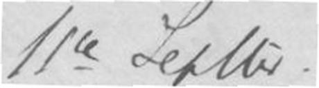11te Septbr
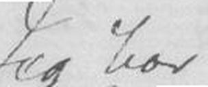Jeg har
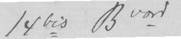14 bis B vor
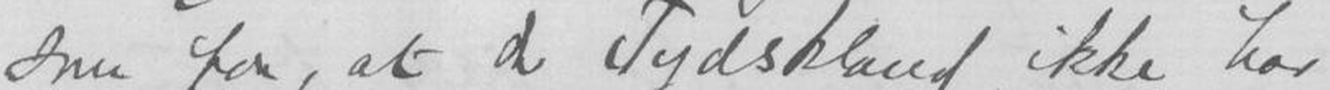som for, at Tydskland ikke har
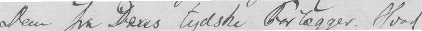Dem fra Deres tydske Forlægger. Hvad
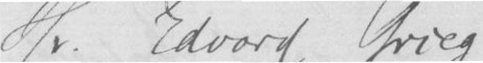Hr. Edvard Grieg
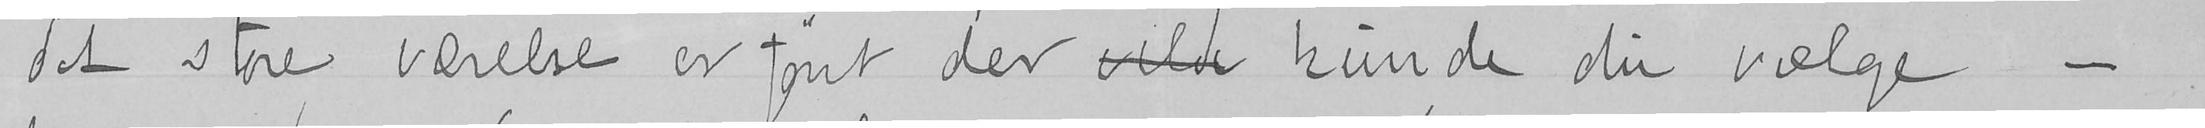det store værelse er tømt der vilde kunde du vælge -
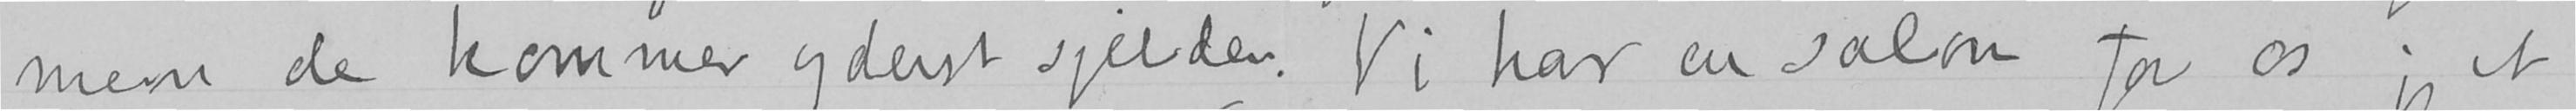men de kommer yderst sjelden. Vi har en salon for os og et
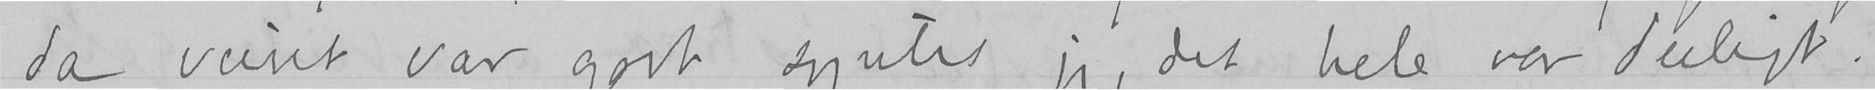da veiret var godt syntes jeg, det hele var deiligt.
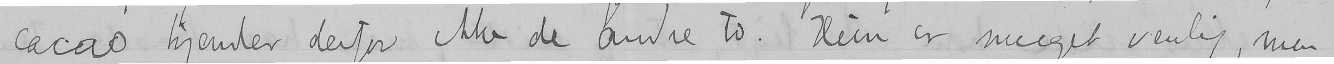cacao kjender derfor ikke de andre to. Hun er meget venlig, men
